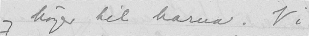og bøger til barna. Vi
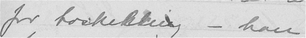for toskilling - han
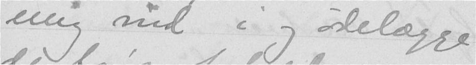mig ind i og ødelægge
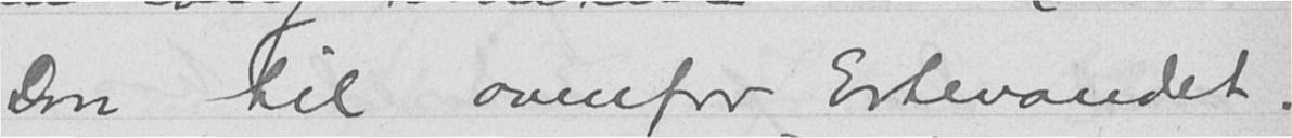Don til ovenfor Ertevandet.
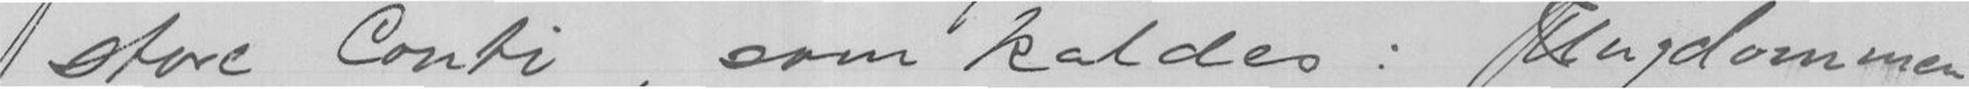store Conti, som kaldes: Ungdommen
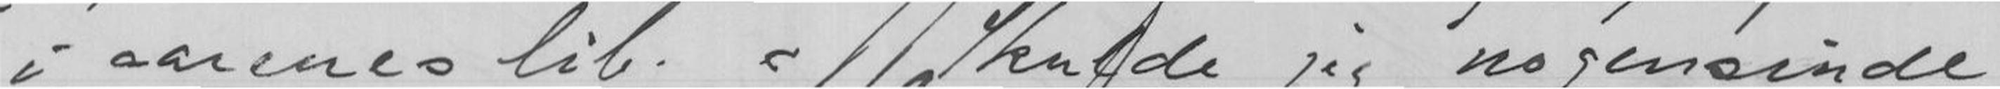i aarenesløb. - Skulde jeg nogensinde
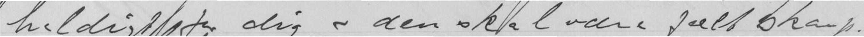heldigere for dig - den skal være fuldt skarp.
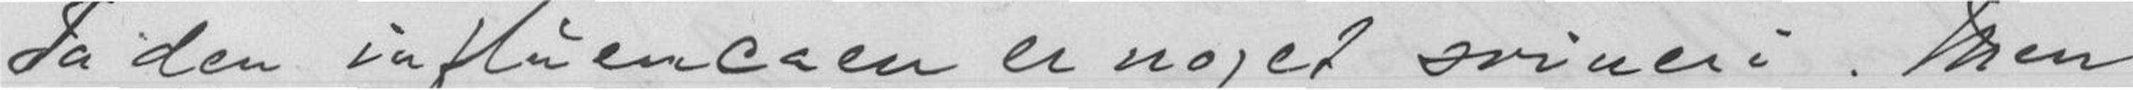Ja den influencaen er noget svineri. Men
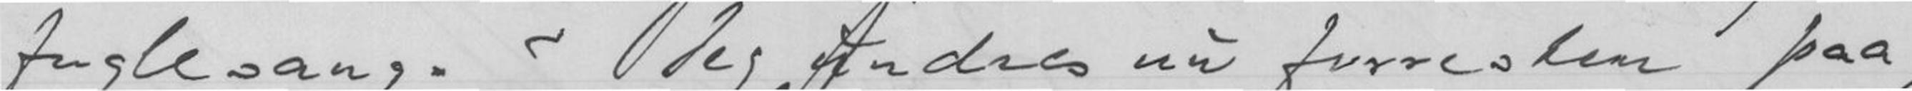fuglesang. - Jeg undres nu forresten paa,
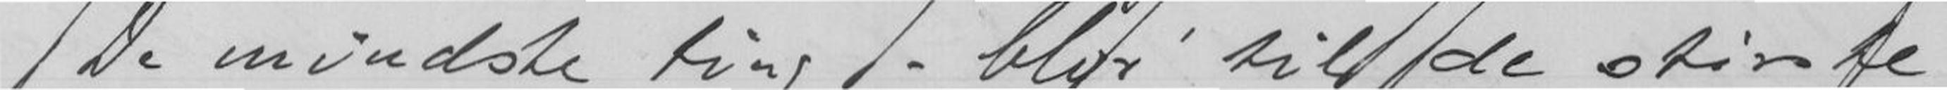De mindste ting - blir til de største
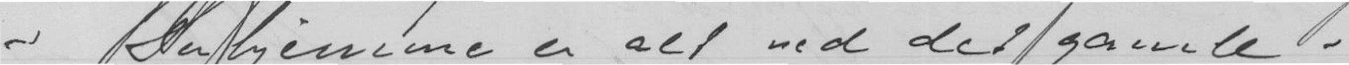- Her hjemme er alt ved det gamle -
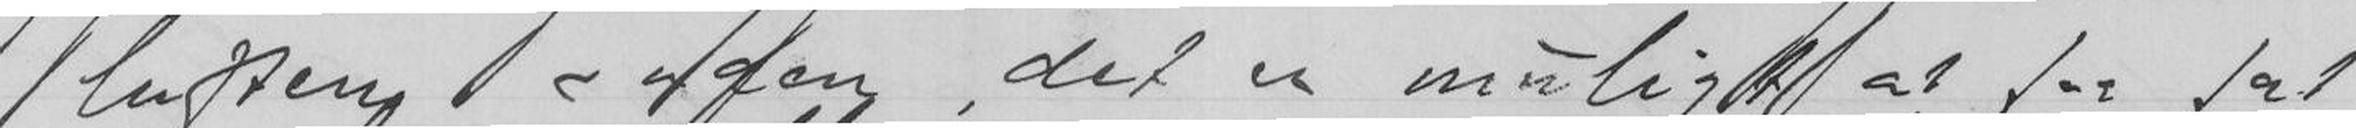luften - uden det er muligt at faa fat
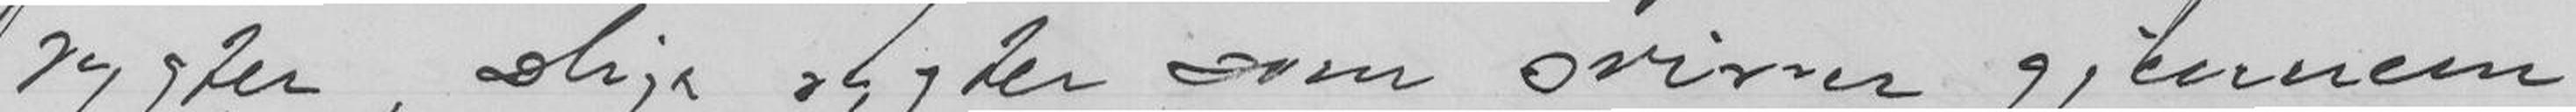rygter, slige rygter som svirrer gjennem
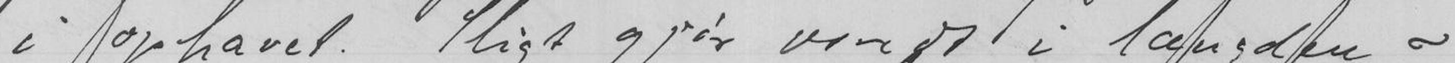i ophavet. Sligt gjør vondt i længden -
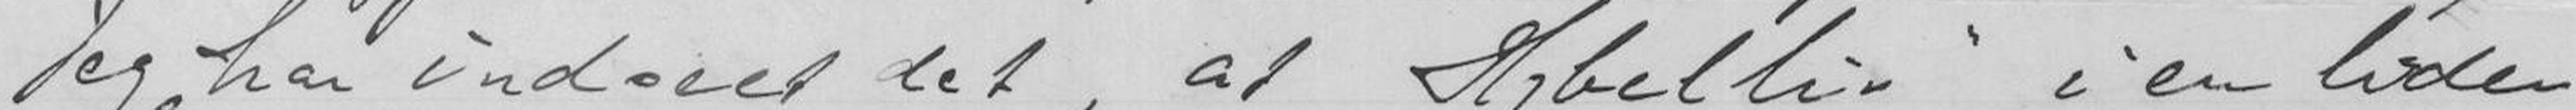Jeg har indseet det, at Hybelliv i en liden
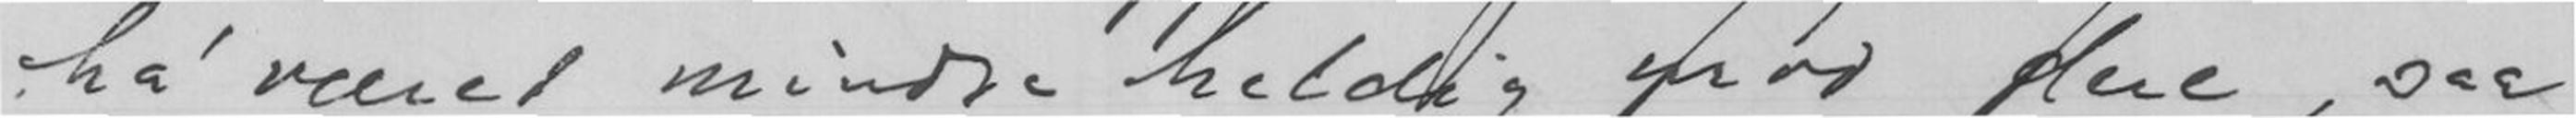ha' været mindre heldig mod dere, sa
